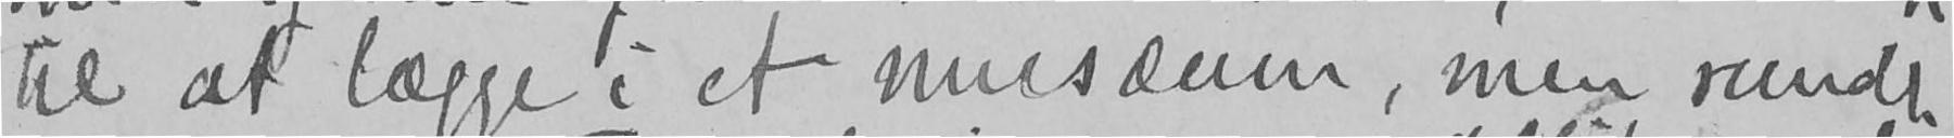til at lægge i et musæum, men runde-
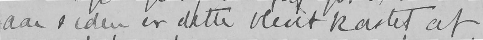aar siden er dette blevet kastet af
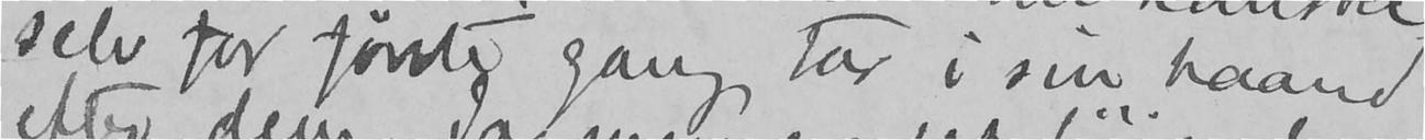selv for første gang tar i sin haand
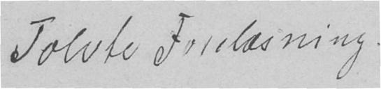Tolvte Forelæsning.
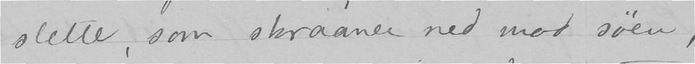slette, som skraaner ned mod søen,
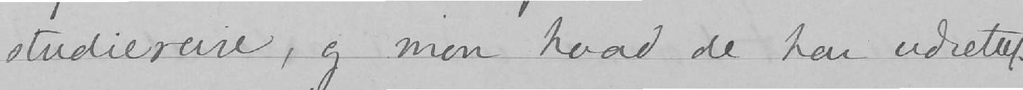studiereise, og mon hvad de har udrettet.
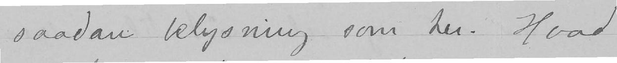saadan belysning som her. Hvad
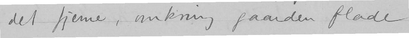det fjerne, omkring gaarden flade
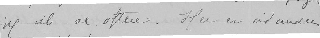jeg vil se oftere. Her er vidunder-
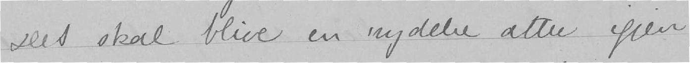Det skal blive en nydelse atter igjen
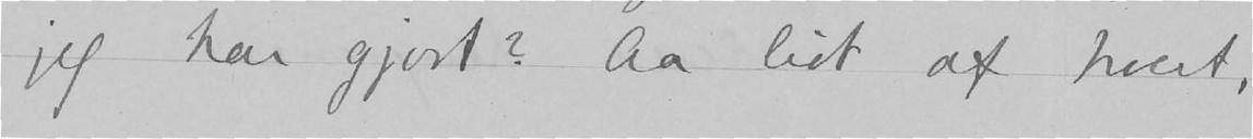jeg har gjort? Aa lidt af hvert,
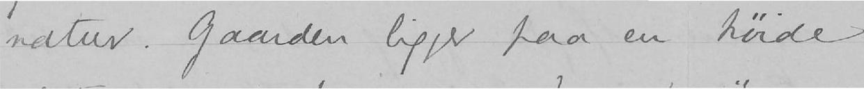natur. Gaarden ligger paa en høide
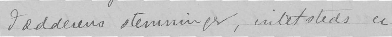Jædderens stemninger, intetsteds er
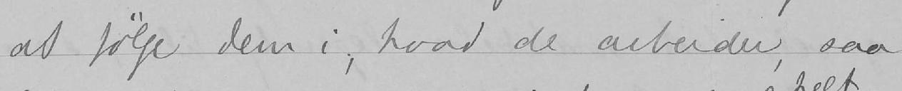at følge dem i, hvad de arbeider, saa
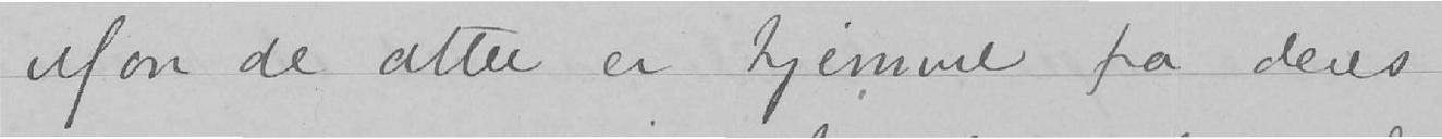Mon de atter er hjemme fra deres
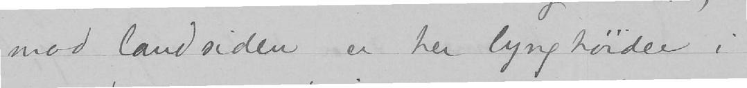mod landsiden er her lynghøider i
